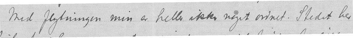Med flytningen min er heller ikke noget ordnet. Stedet her
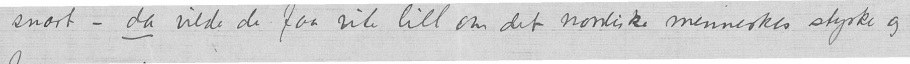snart – da vilde de faa vite litt om det nordiske menneskes styrke og
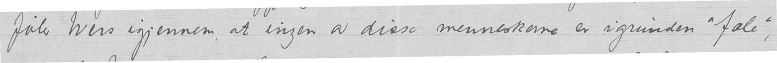føler tvers igjennem, at ingen av disse menneskerne er igrunden "fæle",
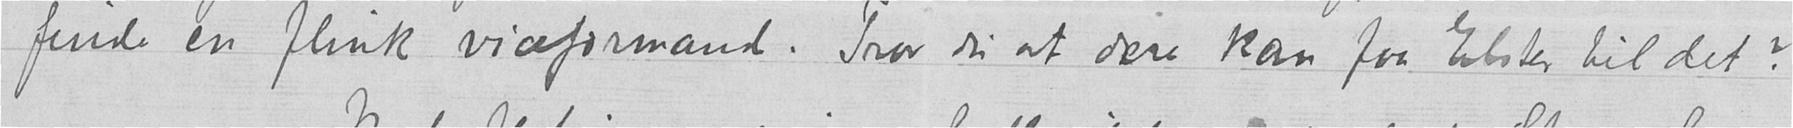finde en flink viceformand. Tror du at dere kan faa Elster til det?
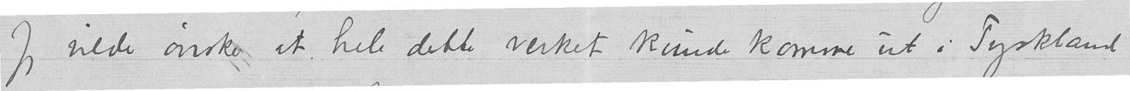Jeg vilde ønske, at hele dette verket kunde komme ut i Tyskland
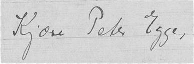Kjære Peter Egge,
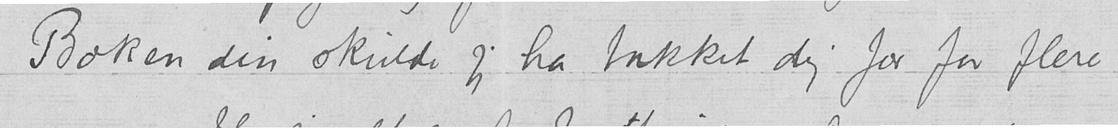Boken din skulde jeg ha takket dig for for flere
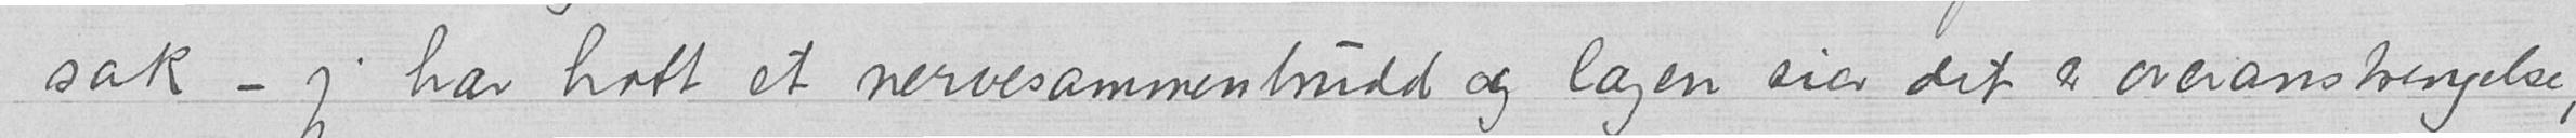sak – jeg har hatt et nervesammenbrudd og lægen sier det er overanstrengelse,
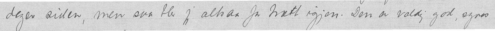dager siden, men saa blev jeg altsaa for trætt igjen. Den er vældig god, synes
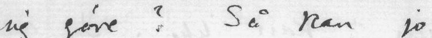sig gøre? Så kan jo
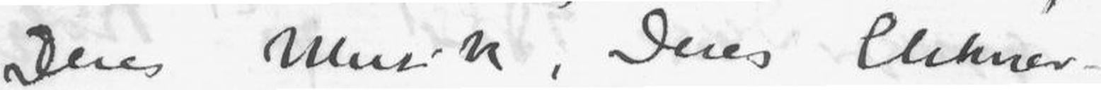Deres Musik, Deres Elskvær-
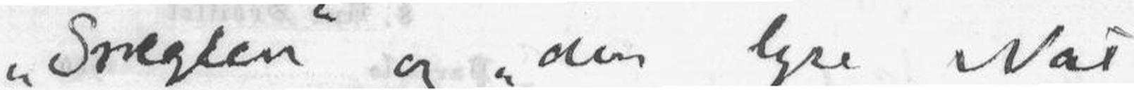Sneglen og "den lyse Nat
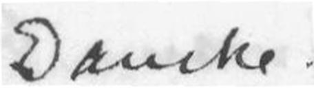Danske.
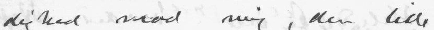dighed mod mig, den lille
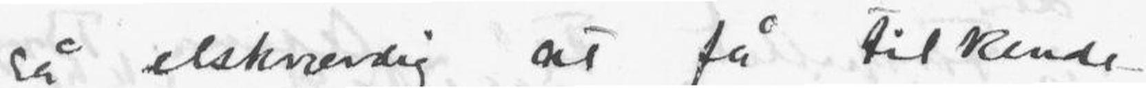så elskværdig at få tilkende-
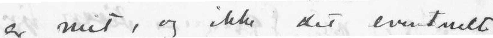er mit, og ikke det eventuelt
